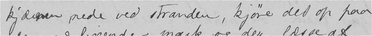kjærren nede ved stranden, kjøre det op paa
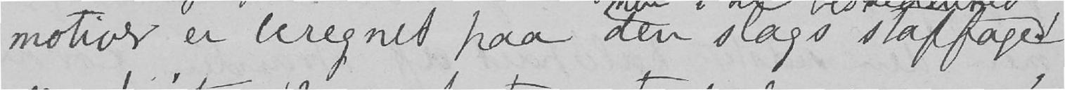motiver er beregnet paa den slags staffage
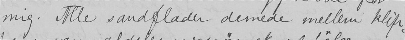mig. Alle sandflader dernede mellem klip-
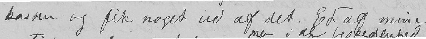kassen og fik noget ud af det. Et af mine
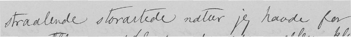straalende storartede natur jeg havde for
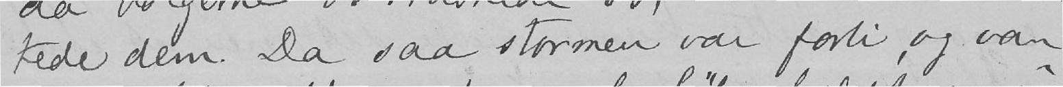tede dem. Da saa stormen var forbi, og van-
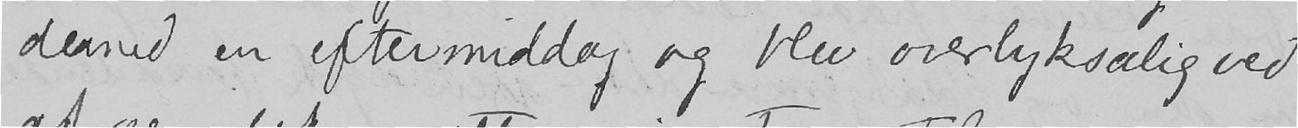derned en eftermiddag og blev overlyksalig ved
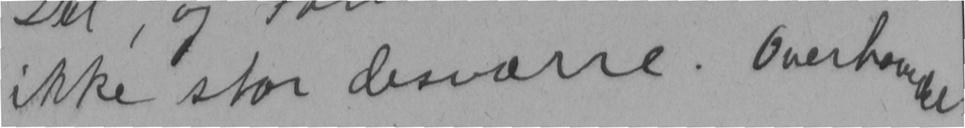ikke stor desværre. Overhovedet
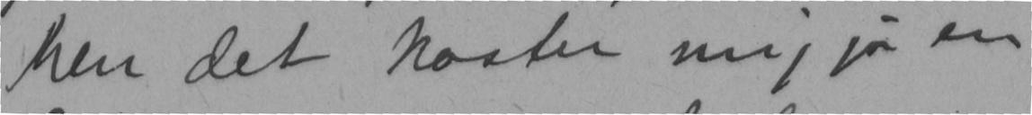Men det koster mig jo en
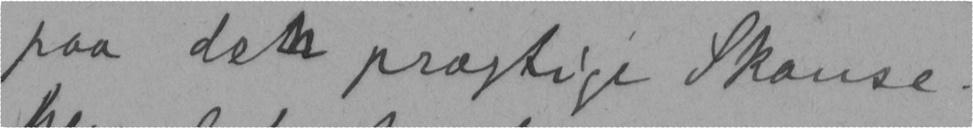paa den prægtige Skanse.
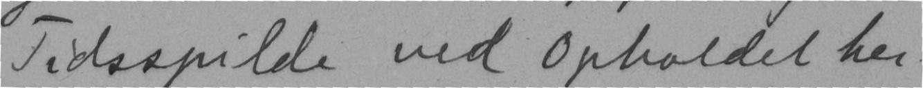Tidsspilde ved Opholdet her.
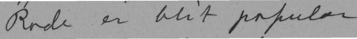Rode er blit populær
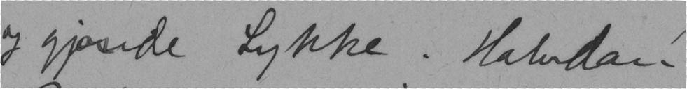og gjorde Lykke. Halvdan
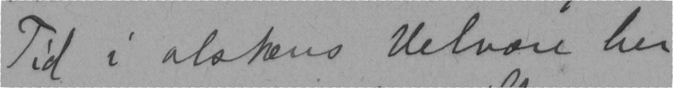Tid i alskens Velvære her
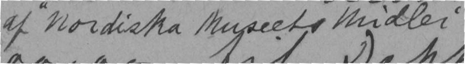af "Nordiska Museets Midler"
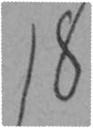18
